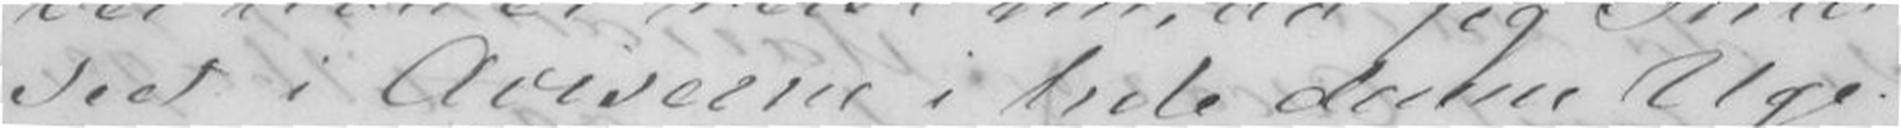seet i Aviserne i hele denne Uge.
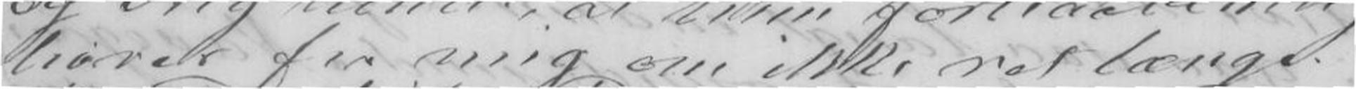hører fra mig om ikke ret længe.
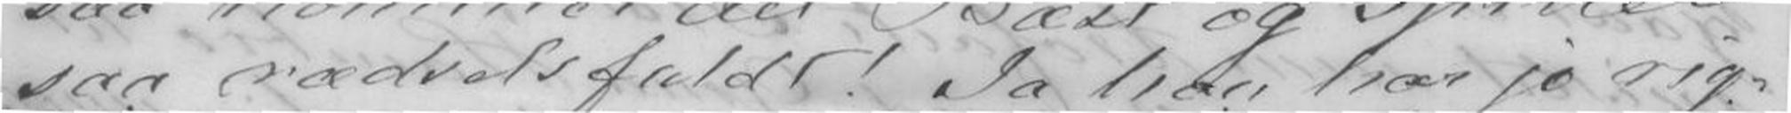saa rædselfuldt! Ja han har jo rig-
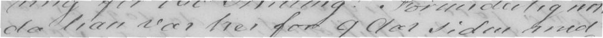da han var her for 9 Aar siden med
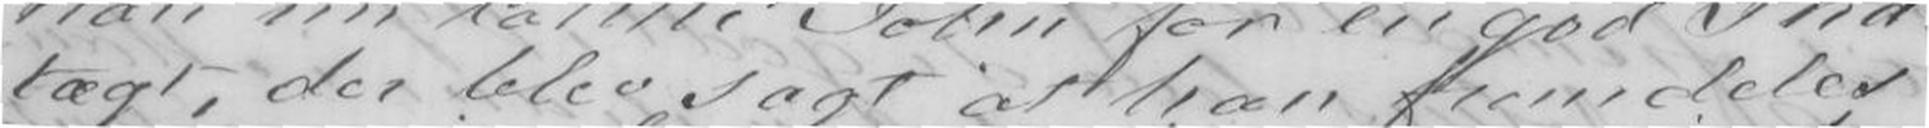tægt, der blev sagt at han fremdeles
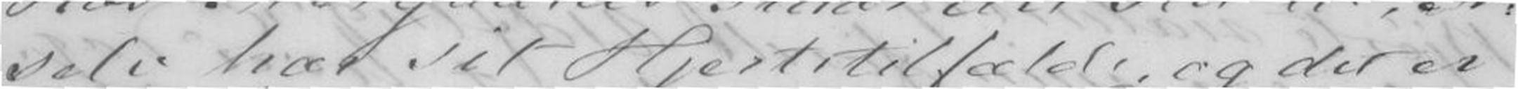selv har sit Hjertetilfælde, og det er
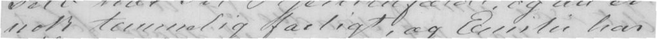nok temmelig farligt, og Emilie har
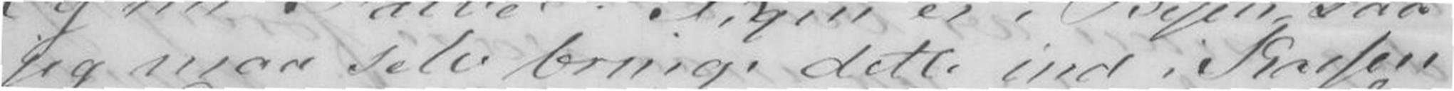jeg maa selv bringe dette ind i Kassen
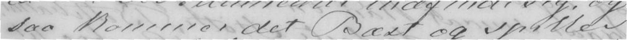saa kommer det Bæst og spiller
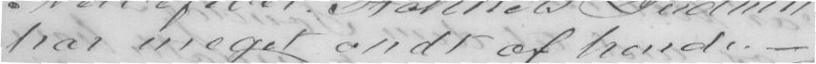har meget ondt af hende. -
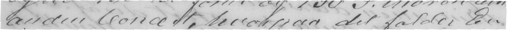anden Concerte hvorpaa det falder En
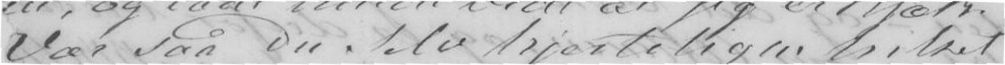Vær saa Du Selv hjerteligen hilset
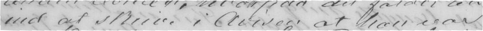ind at skrive i Avisen at han var
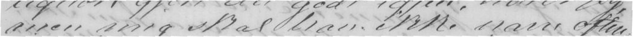men mig skal han ikke narre oftere;
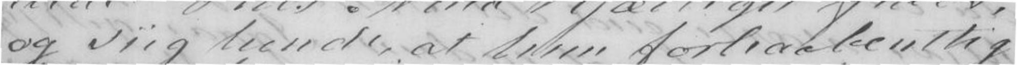og siig hende, at hun forhaabentlig
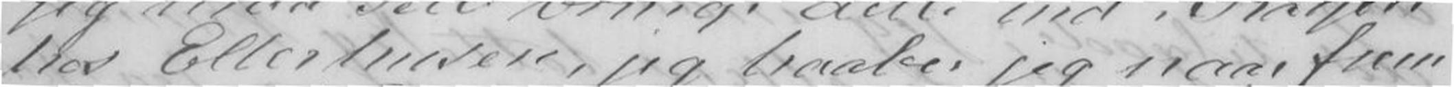hos Ellerhusen, jeg haaber jeg naar frem
